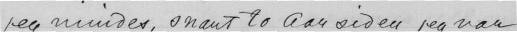jeg mindes, snart to Aarsiden jeg var
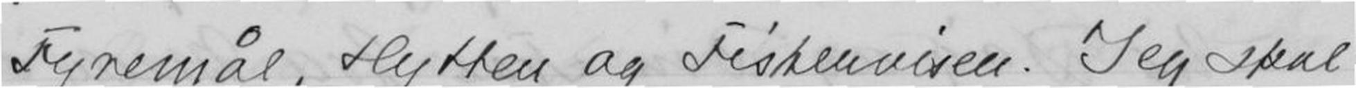Fyremål, Hytten og Feskenvisen. Jeg skal
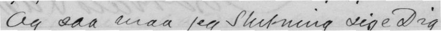Og saa maa jeg slutning sige Dig
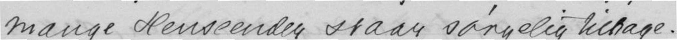mange henseende staar sørgelig tilbage.
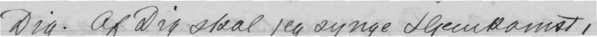Dig. Af Dig skal jeg synge Hjemkomst,
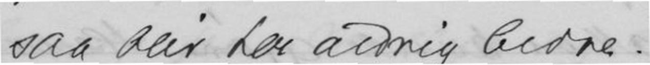saa blir her aldrig bedre.
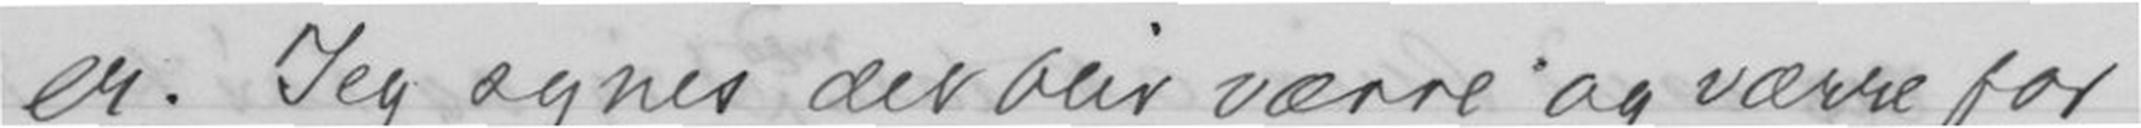er. jeg synes der blir værre og værre for
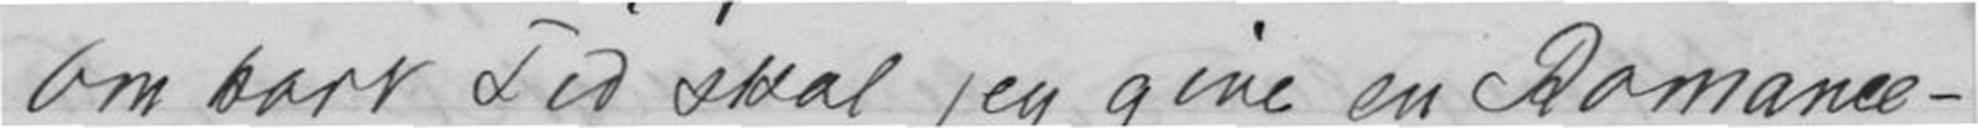Om kort Tid skal jeg give en Romance-
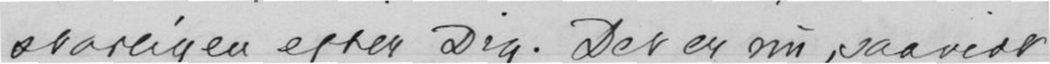storligen efter Dig. Det er nu, saa vidt
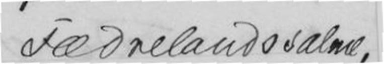Fædrelandssalmæ,
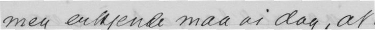men erkjende maa vi dog, at
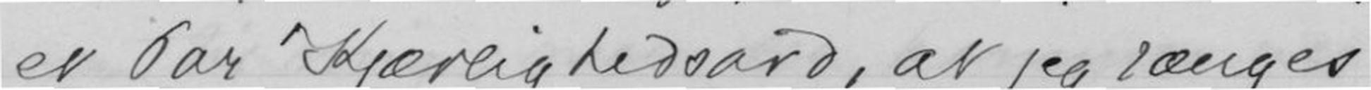et par Kjærlighedsord, at jeg tænger
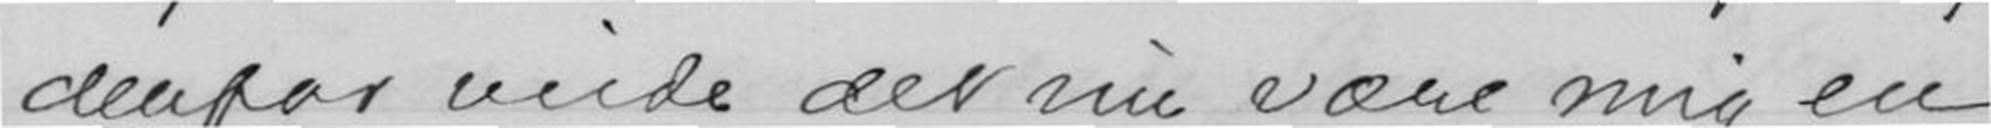derfor vilde det nu være mig en
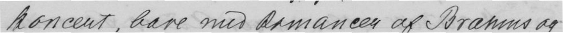koncert, bare med Romancen af Brahms og
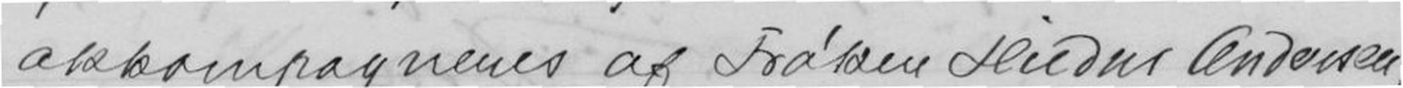akkompagneres af Frøgen Hudne Andersen,
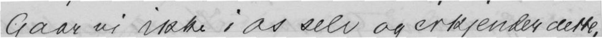Gaar vi ikke i os selv og erkjender dette,
